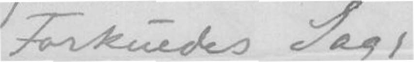Forkuedes Sag,
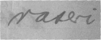raseri
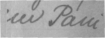en Pani
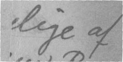lige af
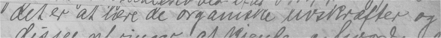"det er "at lære de organiske livskræfter og
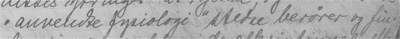"anvendte fysiologi" sted'ae berører og fin-
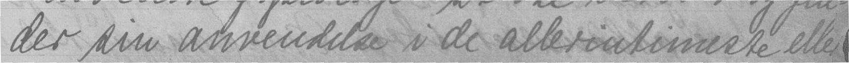der sin anvendelse i de allerintimeste eller
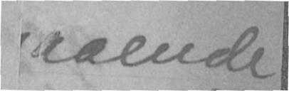aaende
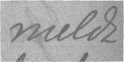meldt
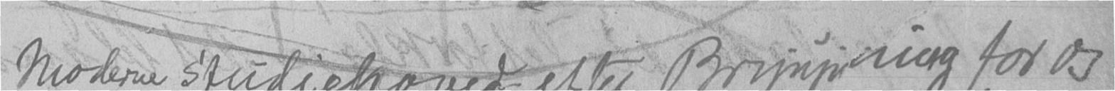Moderne studiehoved efter Brynj  nierg for os
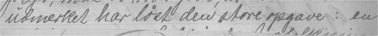udmerket har løst den store opgave: en
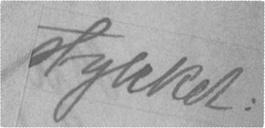stykket:
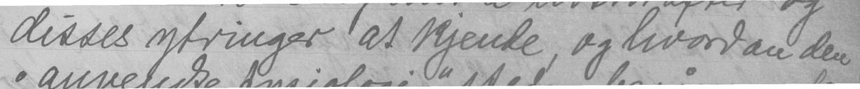disses ytringer at kjende, og hvordan den
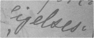ligelses-
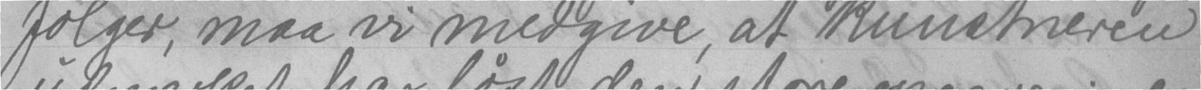følger, maa vi medgive, at kunstneren
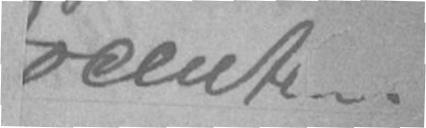ocente -.
